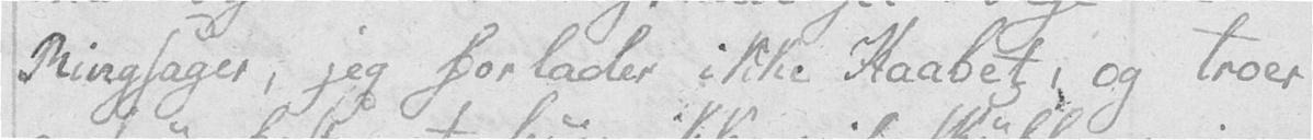Ringsager, jeg forlader ikke Haabet, og troer
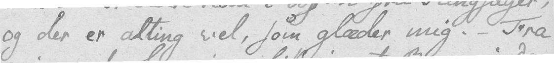og der er alting vel, som glæder mig. – Fra
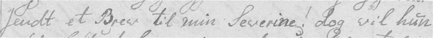sendt et Brev til min Severine! dog vil hun
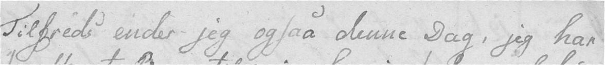Tilfreds ender jeg ogsaa denne Dag, jeg har
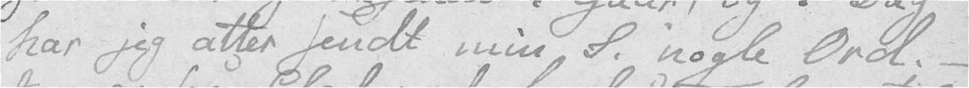har jeg atter sendt min S. nogle Ord. –
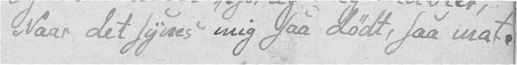Naar det synes mig saa dødt, saa mat.
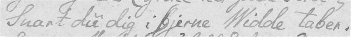Snart du dig i fjerne Vidde taber.
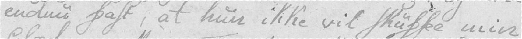endnu fast, at hun ikke vil skuffe min
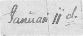Januari 11de
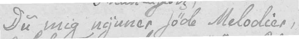Du mig nynner søde Melodier,
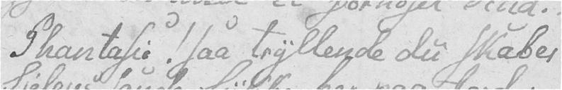Phantasie! saa tryllende du skaber
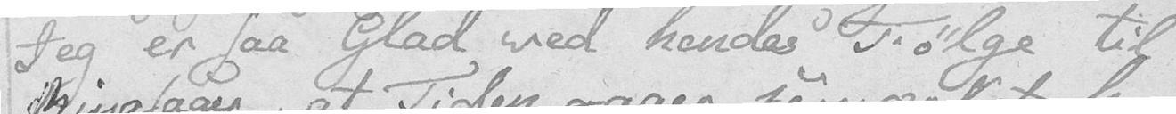Jeg er saa Glad ved hendes Følge til
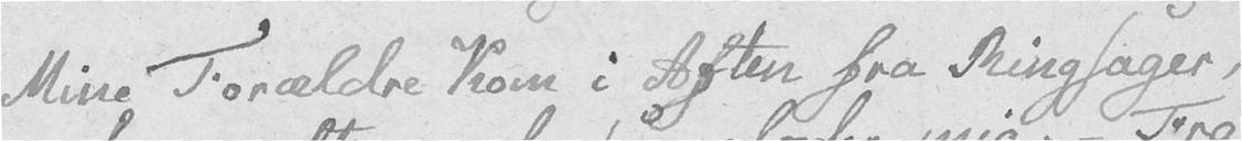Mine Forældre kom i Aften fra Ringsager,
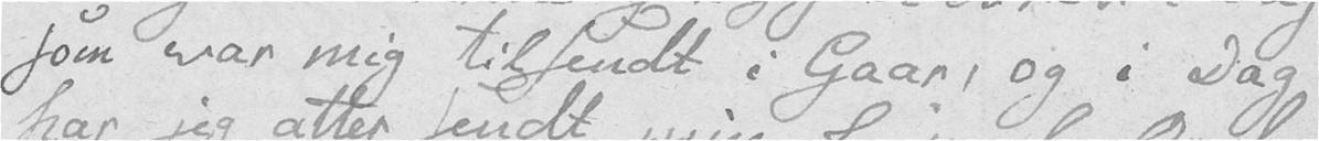som var mig tilsendt i Gaar, og i Dag
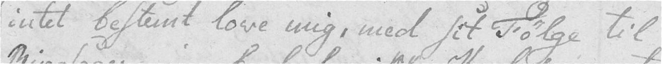intet bestemt love mig, med sit Følge til
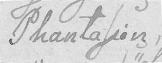Phantasien,
